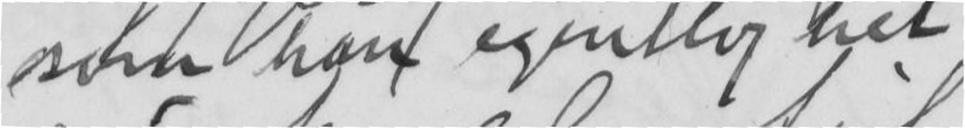som han egentlig het
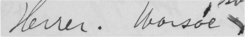Herrer. Worsøe
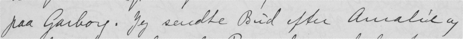paa Garborg. Jeg sendte Bud efter Amalie og
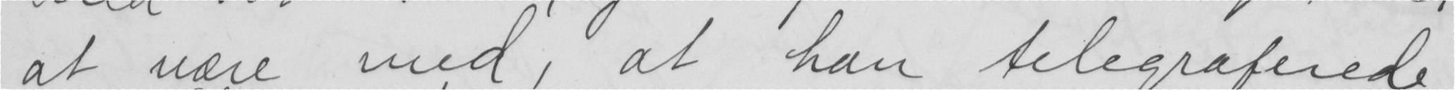at være med, at han telegraferede
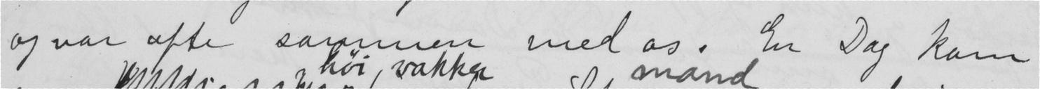og var ofte sammen med os. En Dag kom
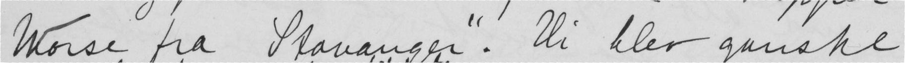Worse fra Stavanger". Vi blev ganske
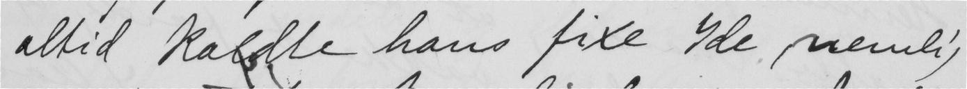altid kaldte hans fixe Ide, nemlig
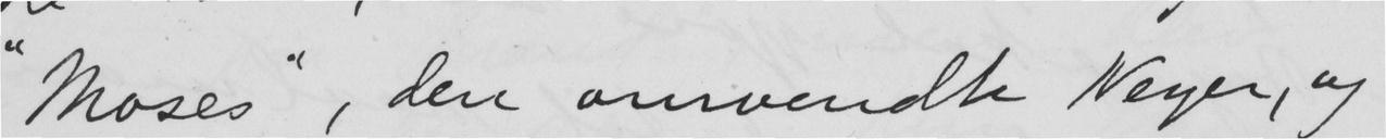"Moses", den omvendte Neger, og
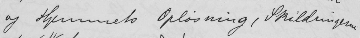og Hjemmets Opløsning, Skildringerne
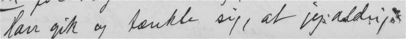Han gik og tænkte sig, at jeg aldrig
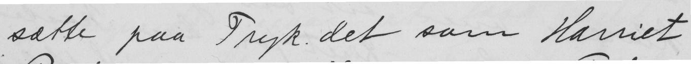sætte paa Tryk det som Harriet
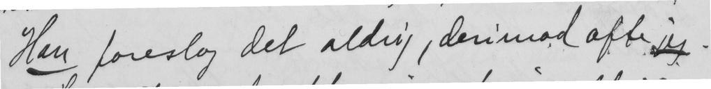Han foreslog det aldrig, derimod ofte jeg.
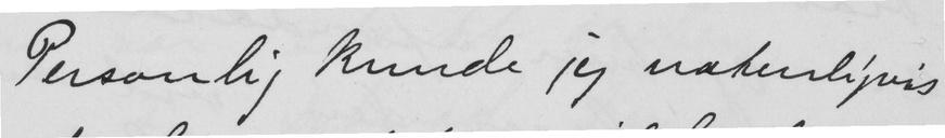Personlig kunde jeg naturligvis
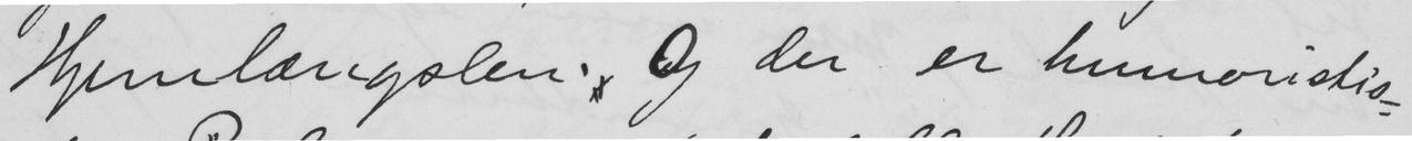Hjemlængslen. Og der er humoristis-
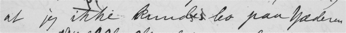at jeg ikke kundes bo paa Jæderen
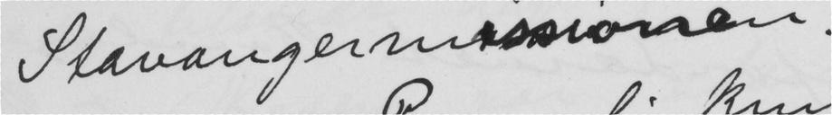Stavangermissionen.
